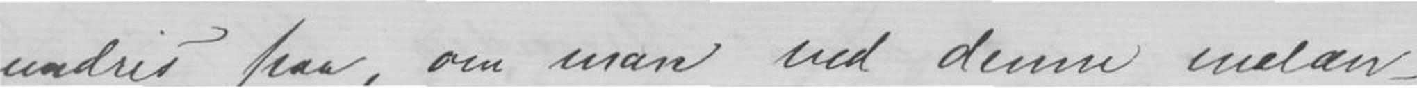undres paa, om man ved denne melan-
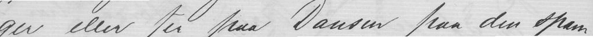ger eller ser paa Dansen paa den span-
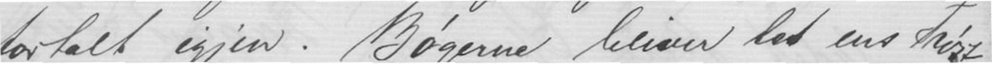fortalt igjen. Bøgerne bliver let ens Trøst.
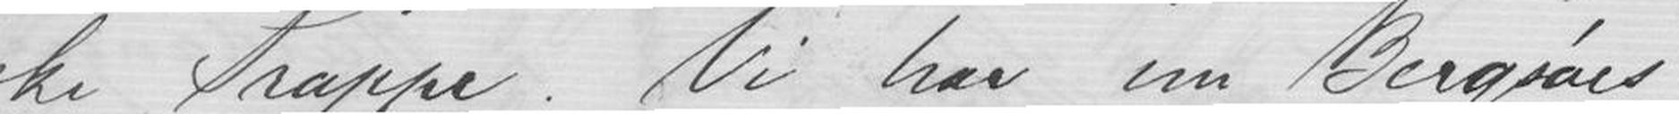ske Trappe. Vi har nu Bergsáes
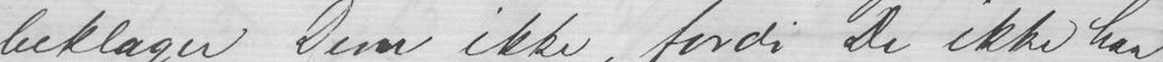beklager Dem ikke, fordi De ikke har
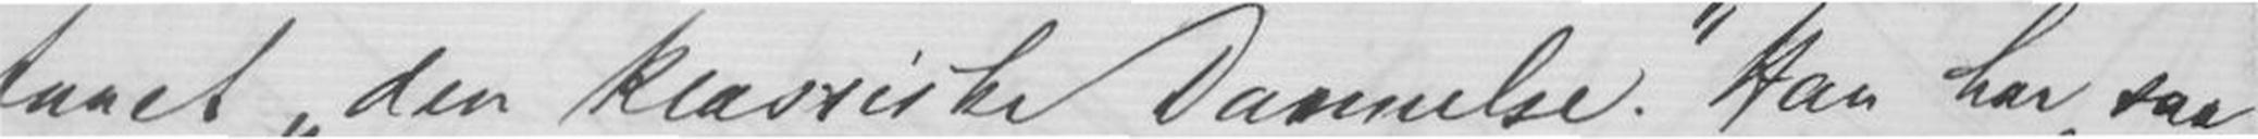faaet "den klassiske Dannelse". Han har saa
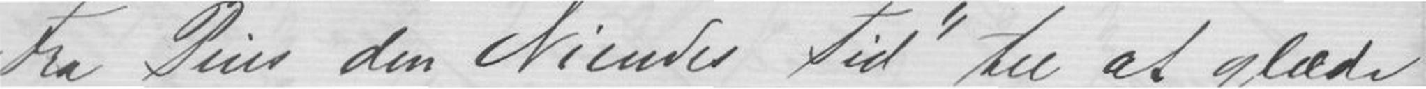"Fra Pins den Niendes Tid" til at glæde
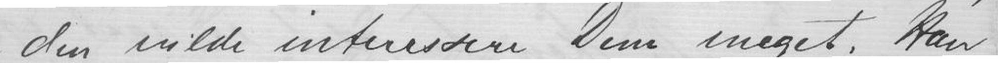- den vilde interessere Dem meget. Han
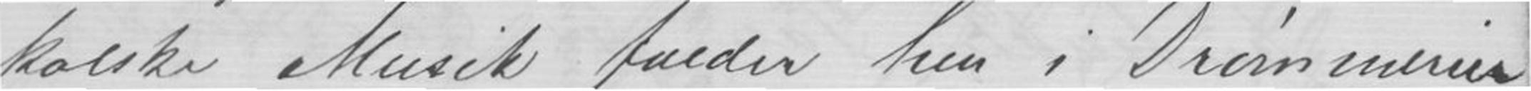kolske Musik falder hen i Drømmerier,
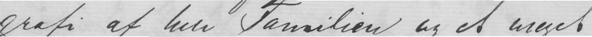grafi af hele Familien og et meget
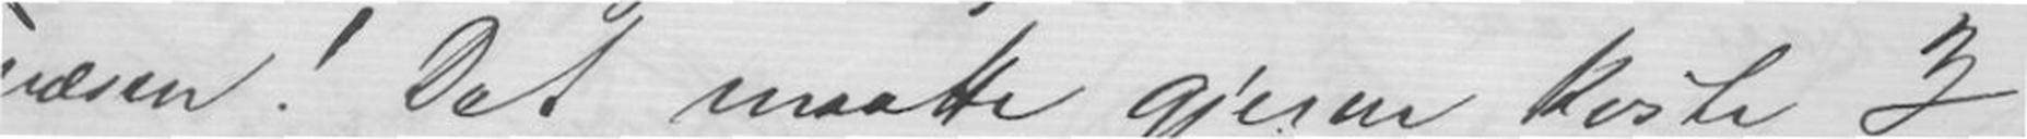væsen! Det maatte gjerne koste 3
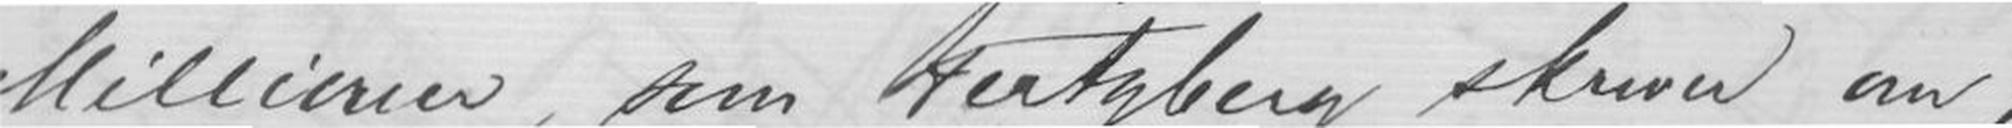Millioner, som Hertzberg skrev om,
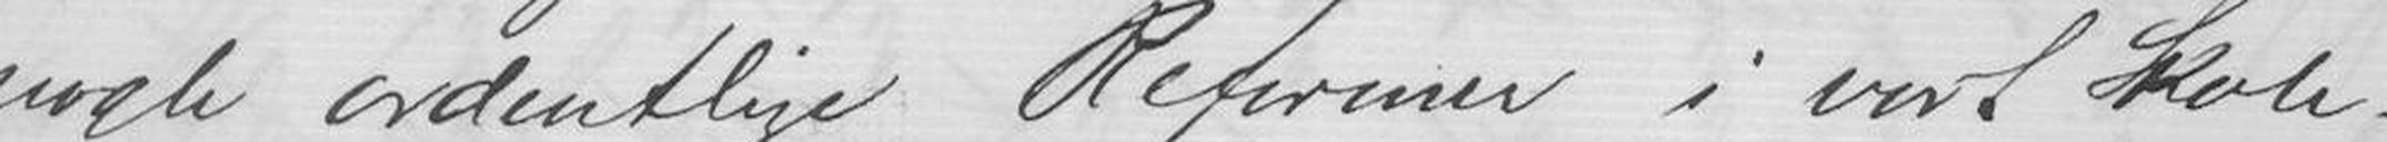nogle ordentlige Reformer i vort Skole-
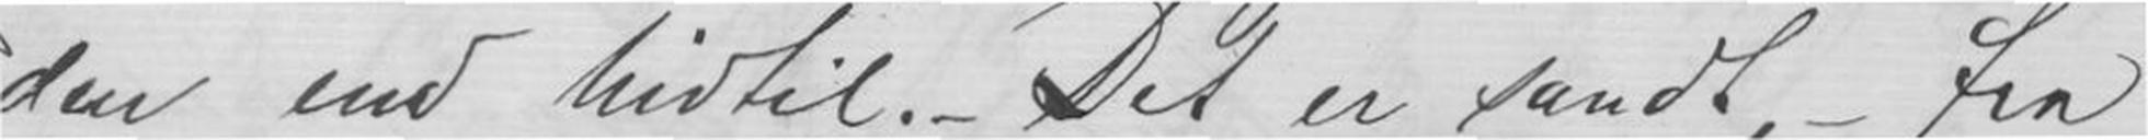den end hidtil. - Det er sandt, - fra
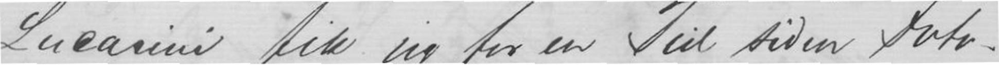Lucarini fik jeg for en Tid siden Foto-
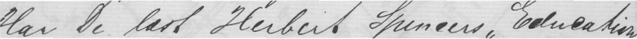Har De læst Herbert Spencers "Education",
